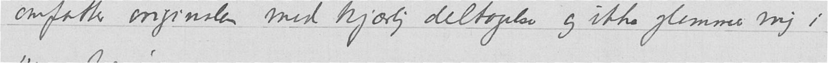omfatter originalen med kjærlig deltagelse og ikke glemmer mig i
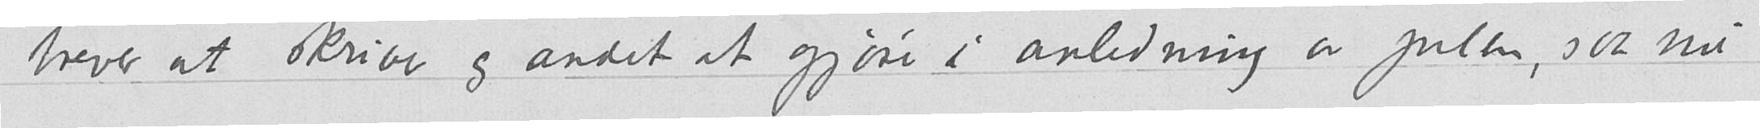brever at skrive og andet at gjøre i anledning av julen, saa nu
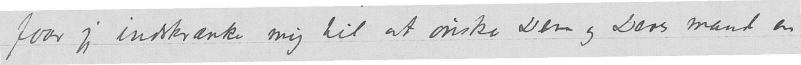faar jeg indskrænke mig til at ønske Dem og Deres mand en
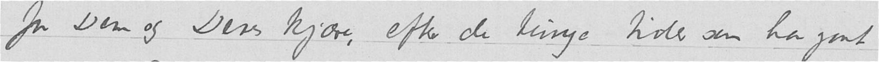for Dem og Deres kjære, efter de tunge tider som har gaat
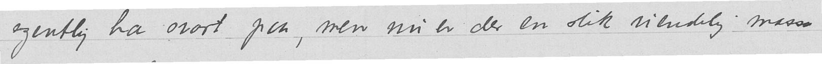egentlig ha svart paa, men nu er der en slik uendelig masse
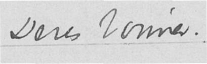Deres bønner.
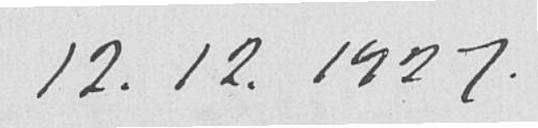12.12.1927.
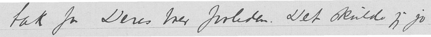tak for Deres brev forleden. Det skulde jeg jo
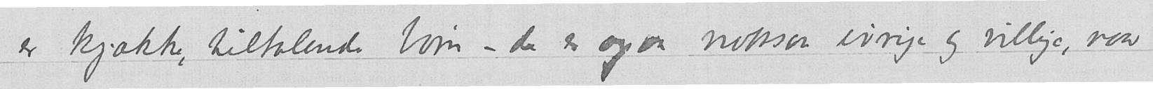er kjække, tiltalende børn - de er ogsaa noksaa ivrige og villige, naar
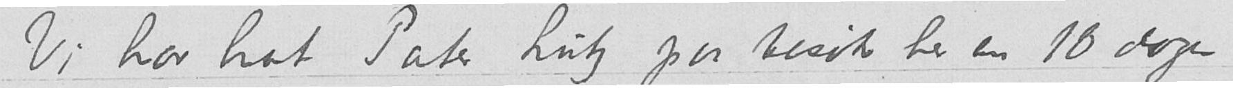Vi har hat Pater Lutz paa besøk her en 10 dages
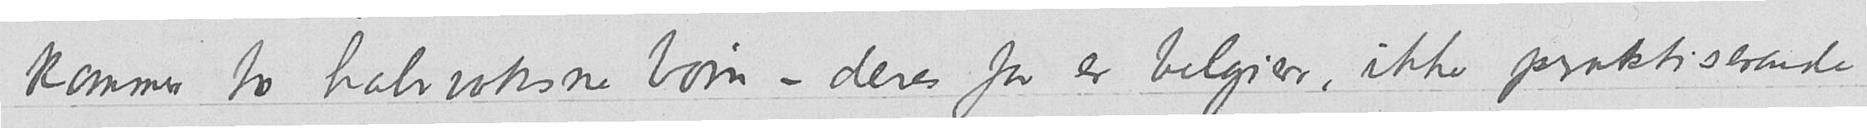kommer to halvvoksne børn - deres far er belgier, ikke praktiserende
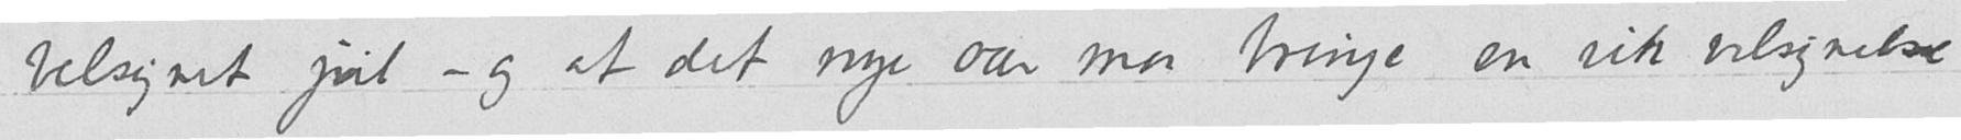velsignet jul – og at det nye aar maa bringe en rik velsignelse
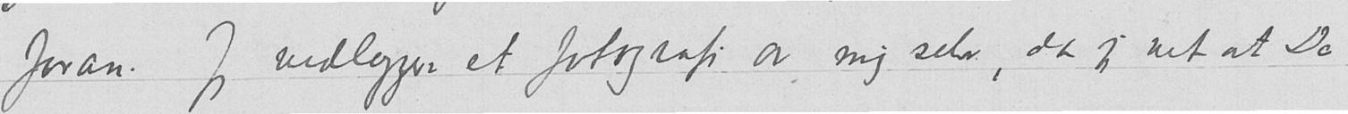foran. Jeg vedlegger et fotografi av mig selv, da jeg vet at De
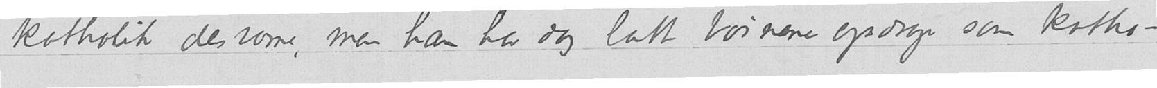katholik desværre, men han har dog latt børnene opdrage som katho-
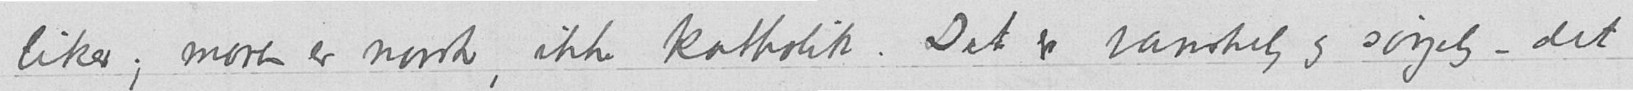liker; moren er norsk, ikke katholik. Det er vanskelig og sørgelig – det
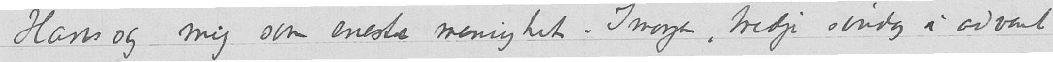Hans og mig som eneste menighet. Imorgen, tredje søndag i advent
